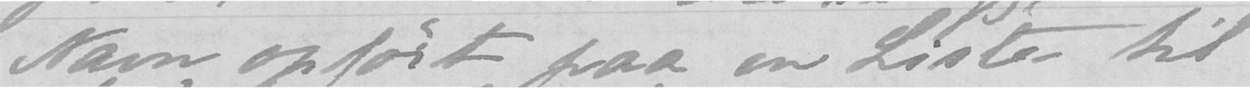Navn opført paa en Liste til
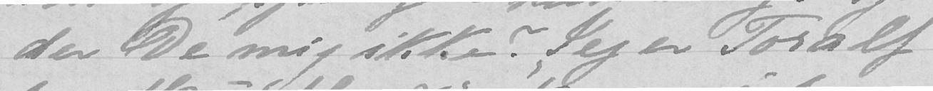der De mig ikke? Jeg er Toralf
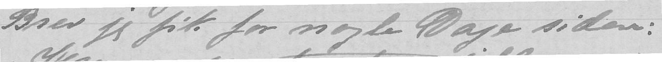Brev jeg fik for nogle Dage siden:
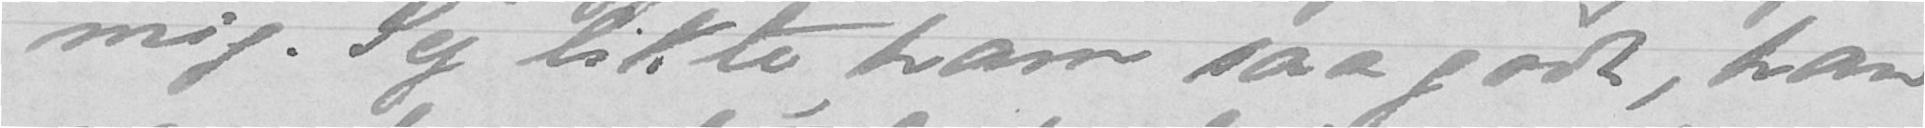mig. Jeg likte ham saa godt, han
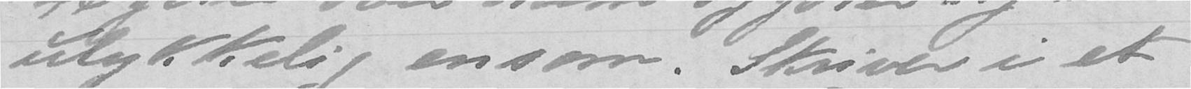ulykkelig ensom. Skriver i et
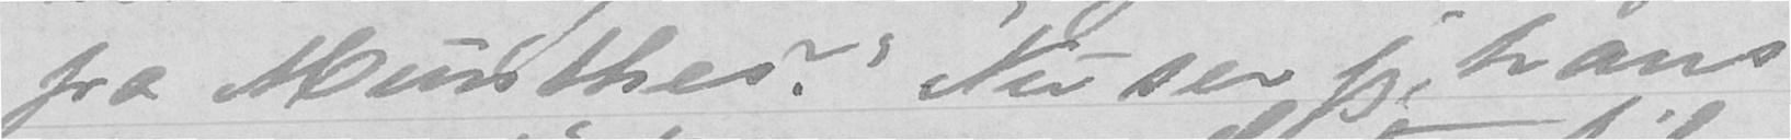fra Munthes! Nu ser jeg, hans
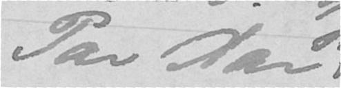Par Aar
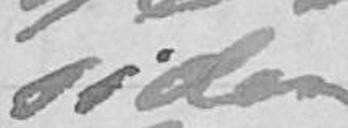siden
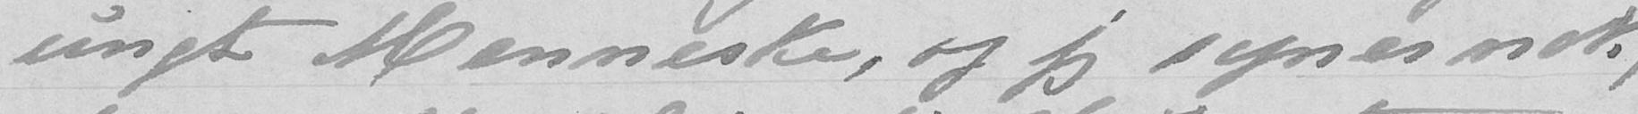ungt Menneske, og jeg synes nok,
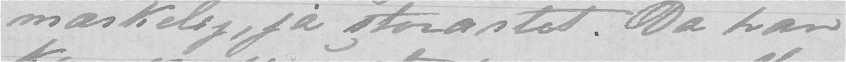mærkelig, ja storartet. Da han
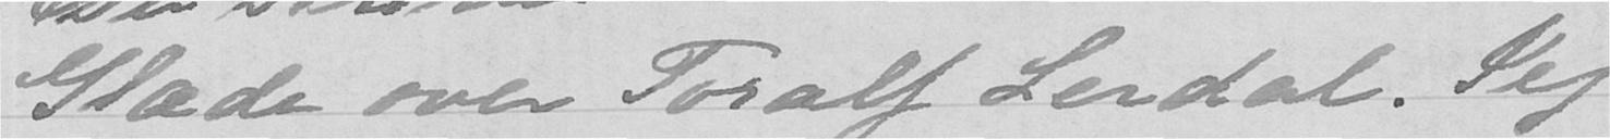Glæde over Toralf Lerdal. Jeg
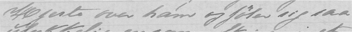Hjerte over ham og føler sig saa
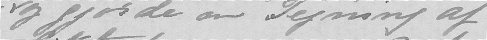og gjorde en Tegning af
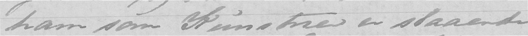ham som Kunstner er slaaende
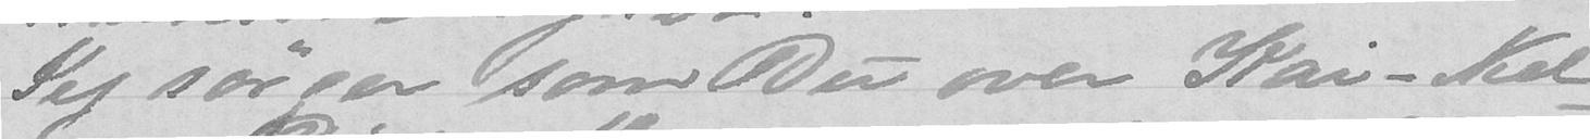Jeg sørger som Du over Kai-Niel-
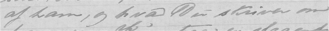af ham, og hvad Du skriver om
